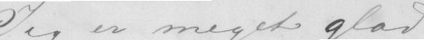Jeg er meget glad
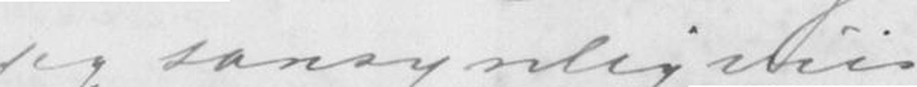jeg sansynligviis
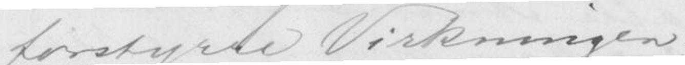forstyrre Virkningen;
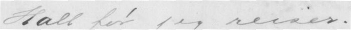Hall før jeg reiser.
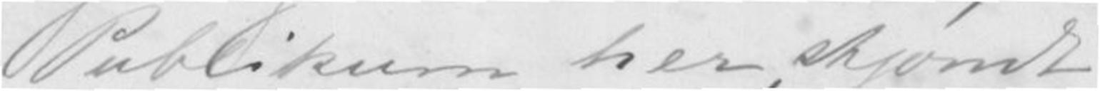Publikum her, skjøndt
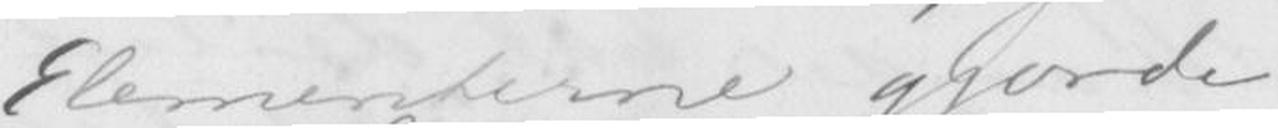Elementerne gjorde
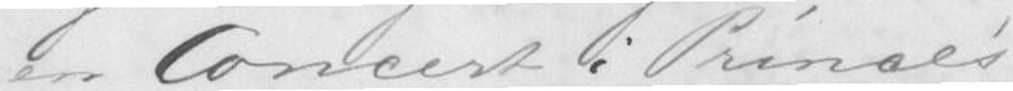en Concert i Prince's
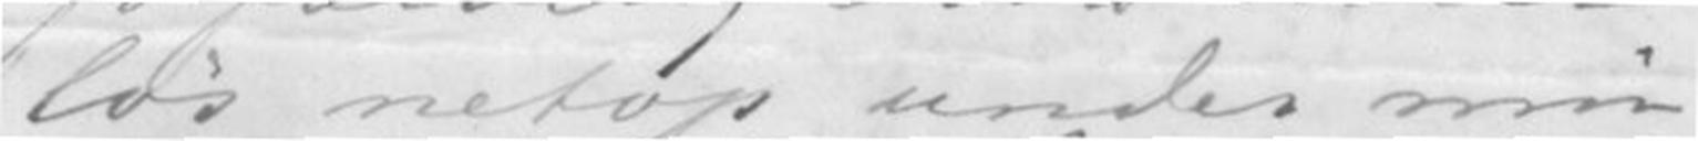løs netop under min
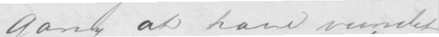gang at have vundet
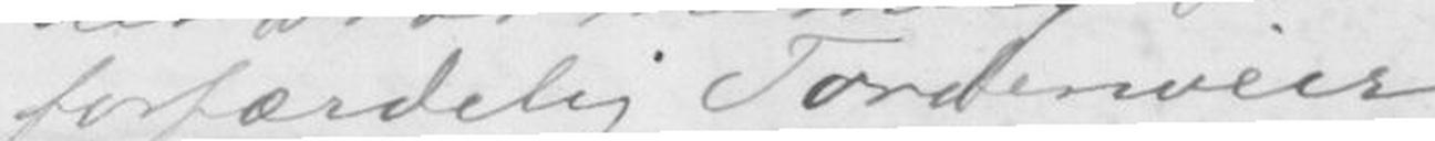forfærdelig Tordenveir
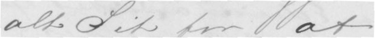alt Sit for at
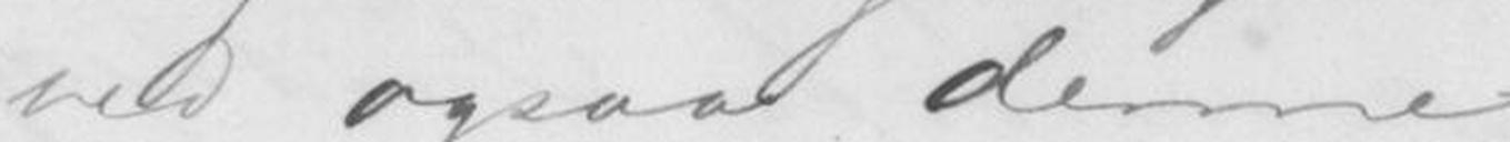ved ogsaa denne
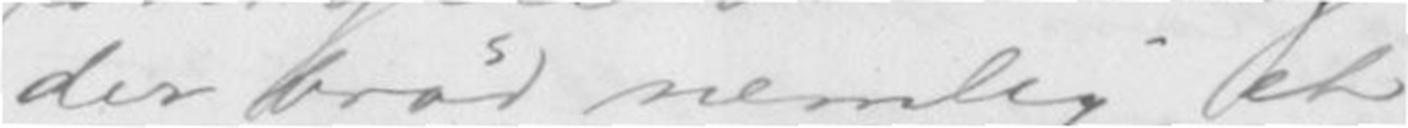der brød nemlig et
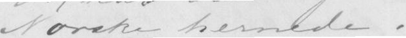Norske hernede i
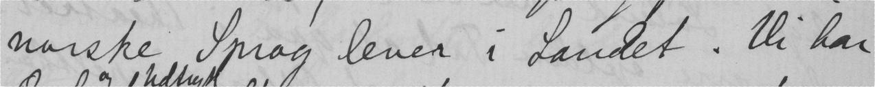norske Sprog lever i Landet. Vi har
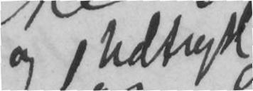og udtryk
